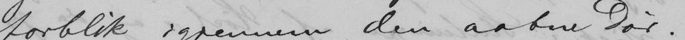torblik igjennem den aabne Dør.
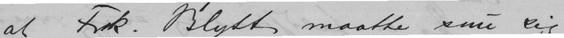at Frk. Blytt maatte snu sig
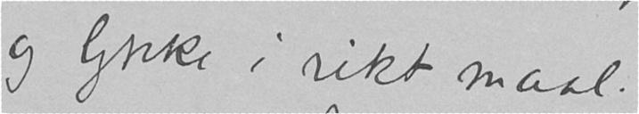og lykke i rikt maal.
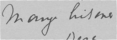Mange hilsener
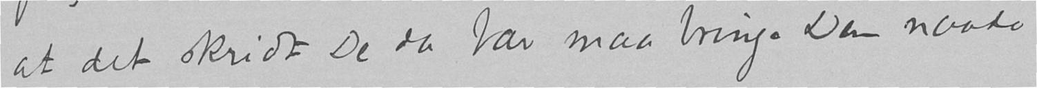at det skridt De da tar maa bringe Dem naade
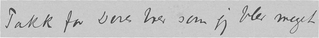Takk for Deres brev som jeg blev meget
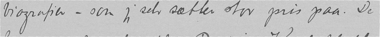biografier - som jeg selv sætter stor pris paa. De
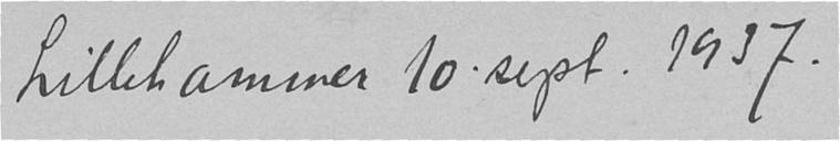Lillehammer 10. sept 1937.
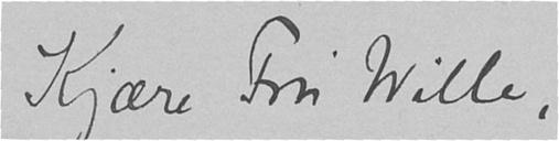Kjære Fru Willer,
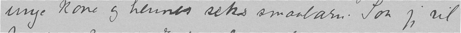unge kone og hennes seks smaabarn. Saa jeg vil
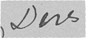Deres
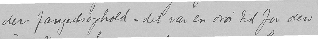ders fængselsophold - det var en drøi tid for den
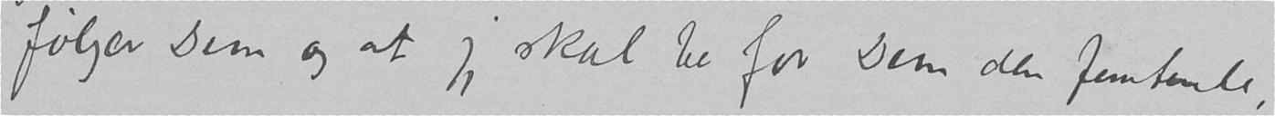følger Dem og at jeg skal be for Dem den femtende,
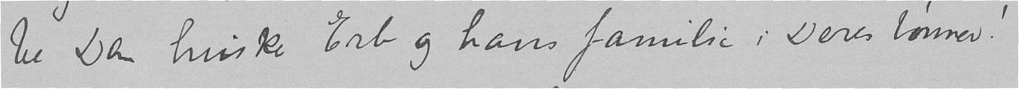be Dem huske Erb og hans familie i Deres bønner!
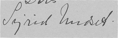Sigrid Undset.
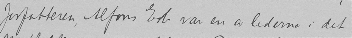forfatteren, Alfons Erb var en av lederne i det
